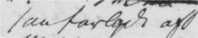saa forladt af
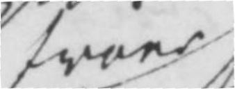troer
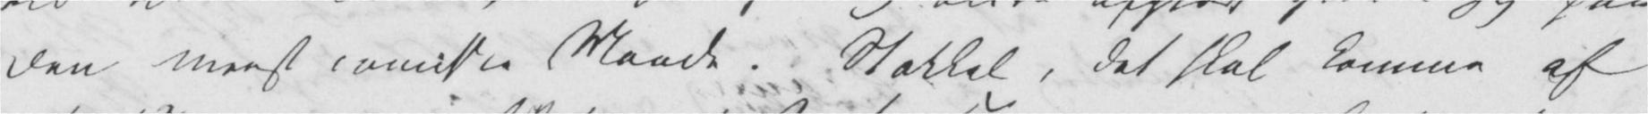den meest comiske Maade. Stakkel, det skal komme af
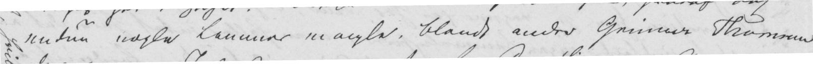endnu nogle Lemmer mangle, blandt andre Grimur Thomsen
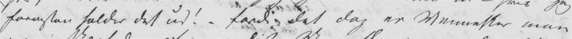forresten holder det ud! – fordi det dog er Mennesker man er
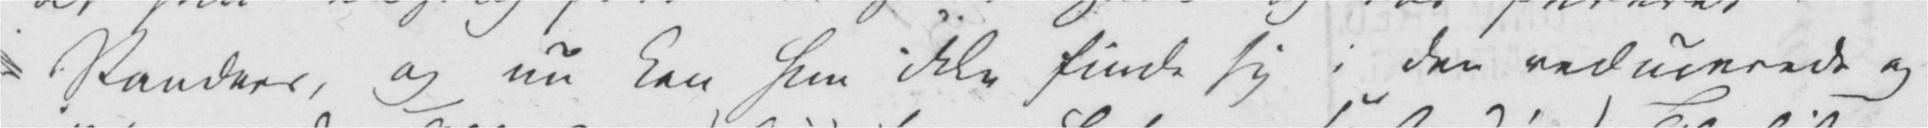Randers, og nu kan hun ikke finde sig i den reducerede og
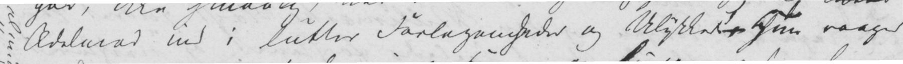Ædelmod ind i lutter Forlegenheder og Ulykker – Hun vaager
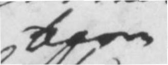bare
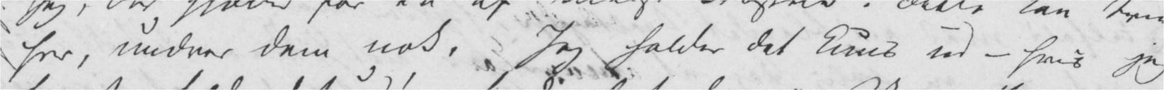her, undrer Dem nok, Jeg holder det kuns ud – hvis jeg
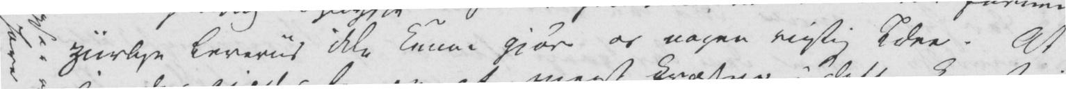ziirlige Leveviis ikke kunne gjøre os nogen rigtig Idee. At
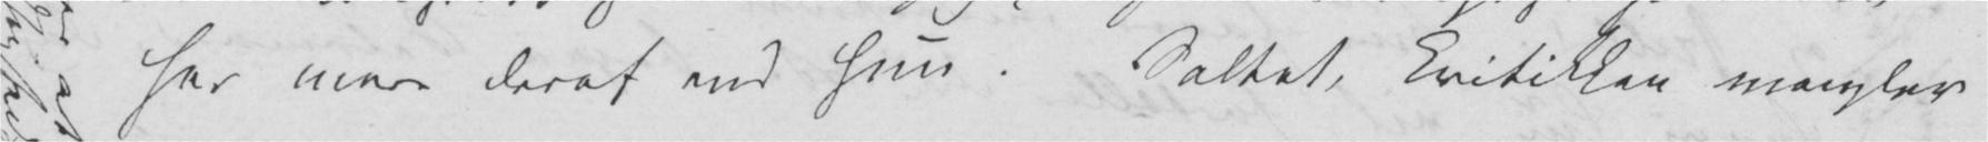har mere deraf end hun. Saltet, Kritikken mangler
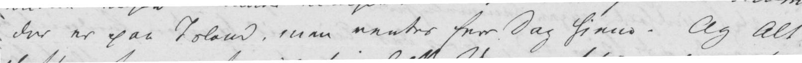der er paa Island, men ventes hver Dag hjem. Og Alt
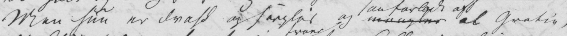Men hun er dvask og sorgløs og mangler al Gratie,
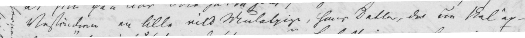Vestindien en lille vild Mulatpige, hans Datter, der nu skal op-
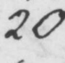20
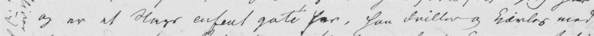og er et Slags enfant gaté her, han driller og kjævles med
